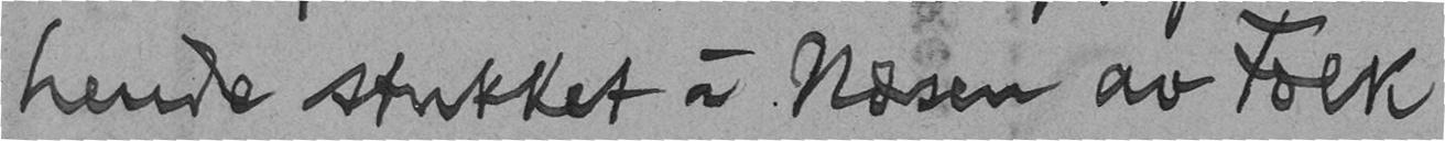hende stukket i Næsen av Folk
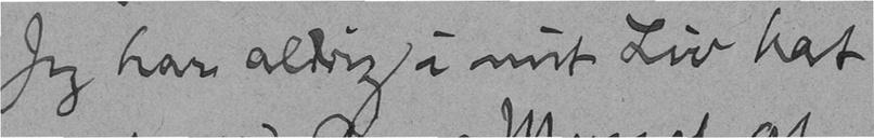Jeg har aldrig i mit Liv hat
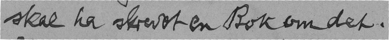skal ha skrevet en Bok om det.
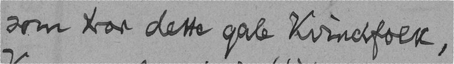som tror dette gale Kvindfolk,
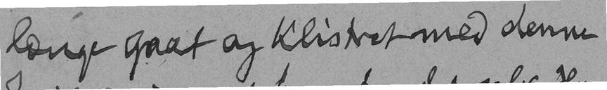længe gaat og klistret med denne
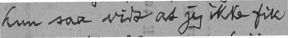hun saa vidt at jeg ikke fik
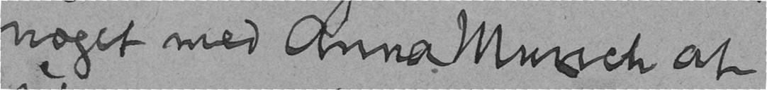noget med Anna Munch at
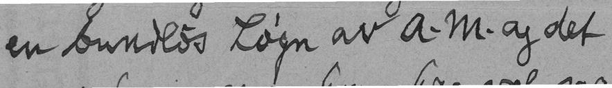en bundløs Løgn av A. M. og det
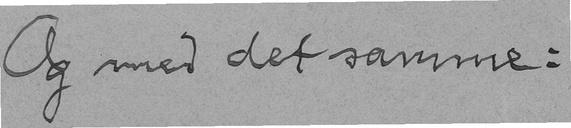Og med det samme:
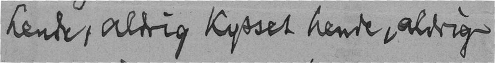hende, aldrig kysset hende, aldrig
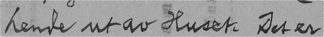hende ut av Huset. Det er
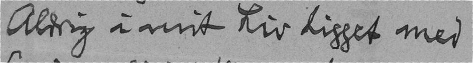Aldrig i mit Liv ligget med
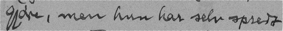gjøre, men hun har selv spredt
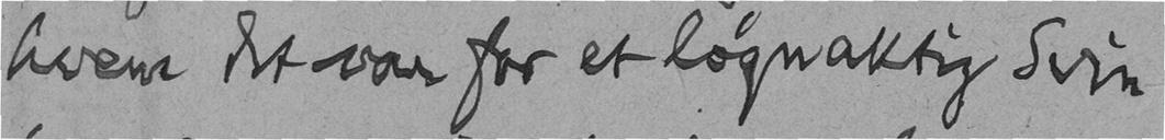hvem det var for et løgnaktig Svin
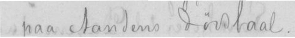paa Aandens Dødsbaal.
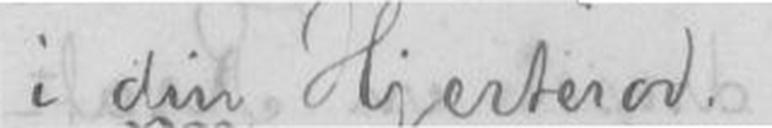i din Hjerterod.
